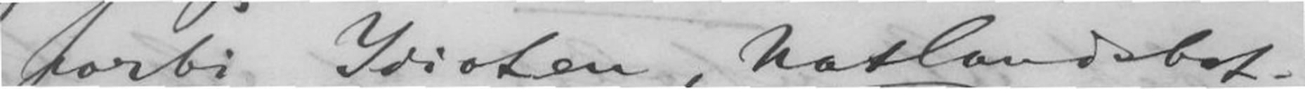forbi Idioten, Natlandsbot-
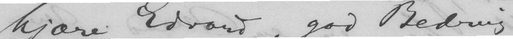kjære Edvard, god Bedring!
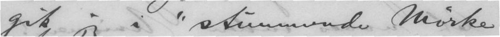gik jeg i "stummende Mørke"
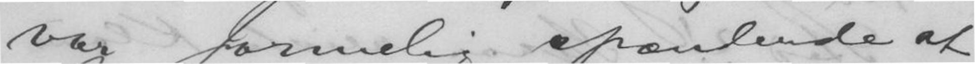var formelig spændende at
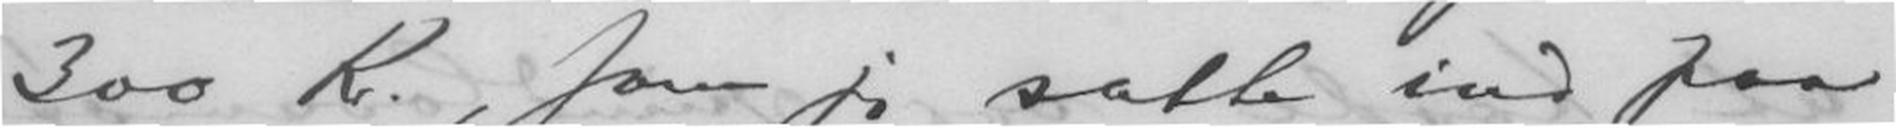300 Kr., som jeg satte ind paa
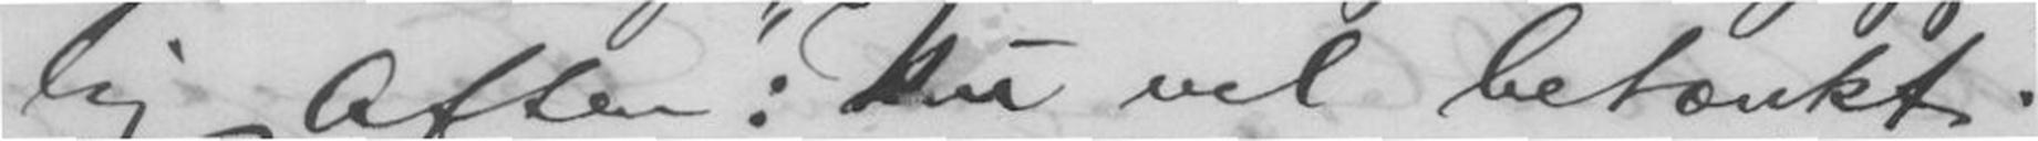lig Aften: "Nu vel betænkt".
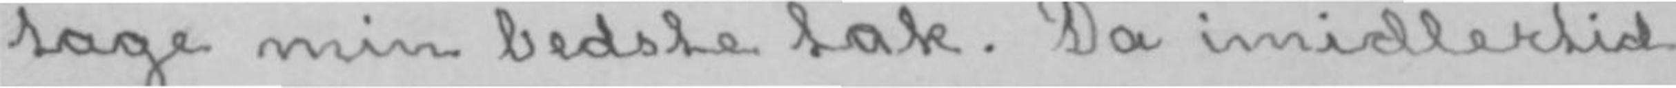tage min bedste tak. Da imidlertid
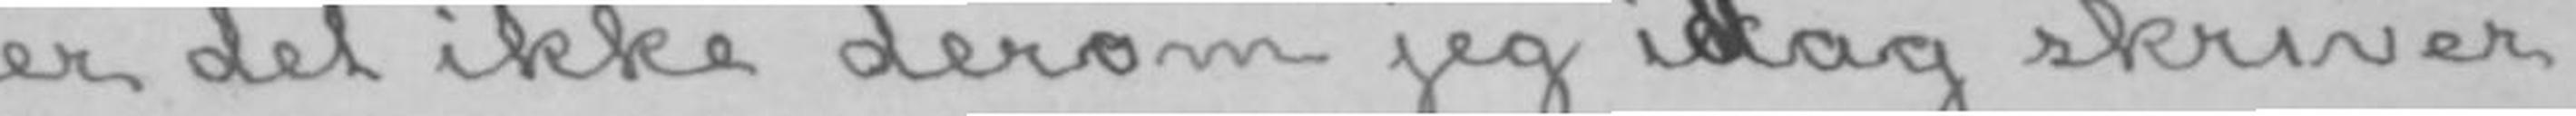er det ikke derom jeg idag skriver
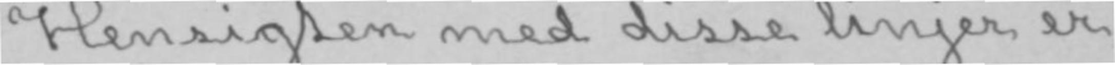Hensigten med disse linjer er
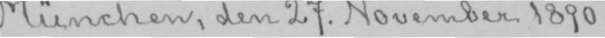München, den 27. November 1890.
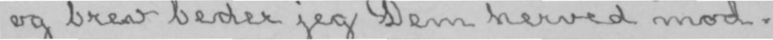og brev beder jeg Dem herved mod-
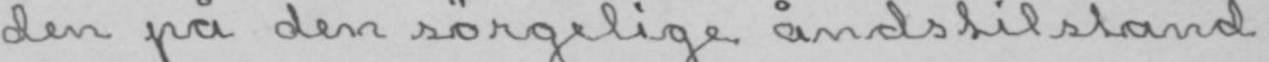den på den sørgelige åndstilstand,
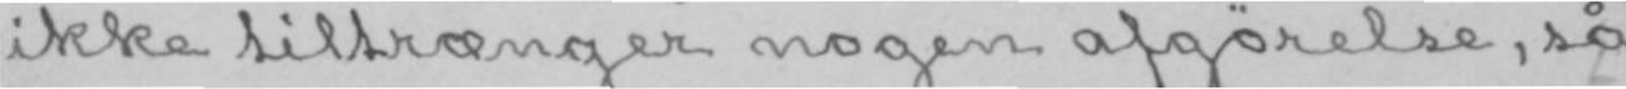ikke tiltrænger nogen afgørelse, så
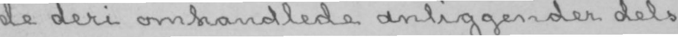de deri omhandlede anliggender dels
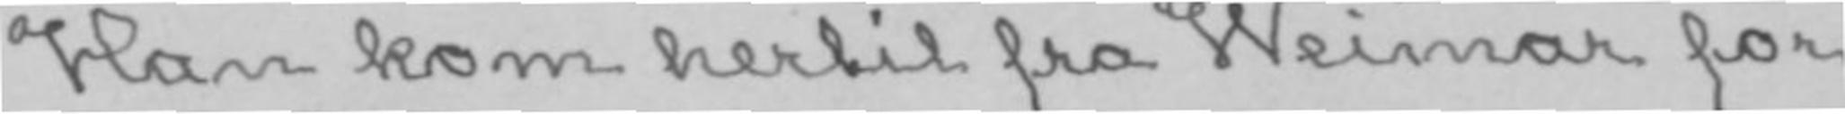Han kom hertil fra Weimar for
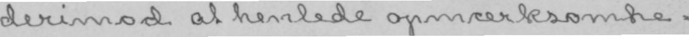derimod at henlede opmærksomhe-
